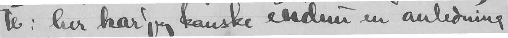te: her har jeg kanske endnu en anledning
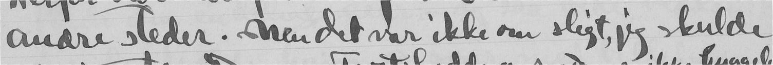andre steder. Men det var ikke om sligt, jeg skulde
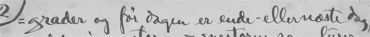-grader og før dagen er ende - eller næste dag,
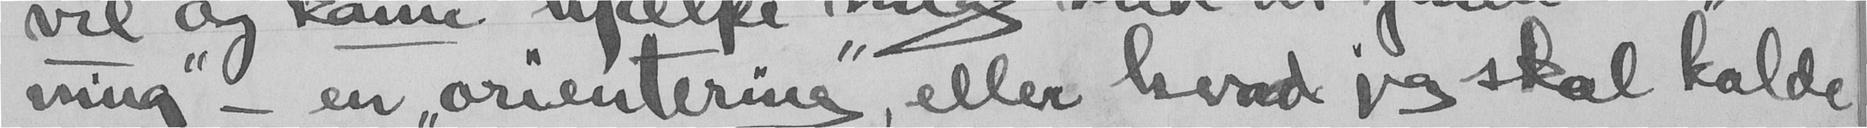ning" - en "orientering", eller hvad jeg skal kalde
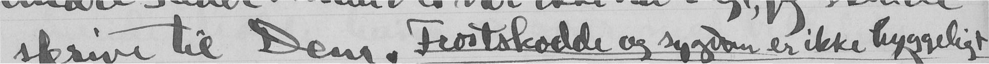skrive til Dem. Frostskodde og sygdom er ikke hyggeligt
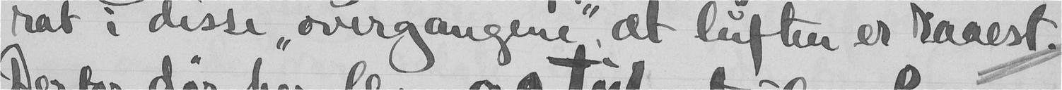rat i disse "overgangene", at luften er raaest.
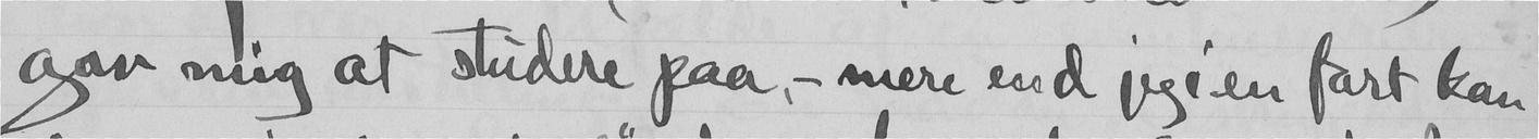gav mig at studere paa, - mere end jeg i en fart kan
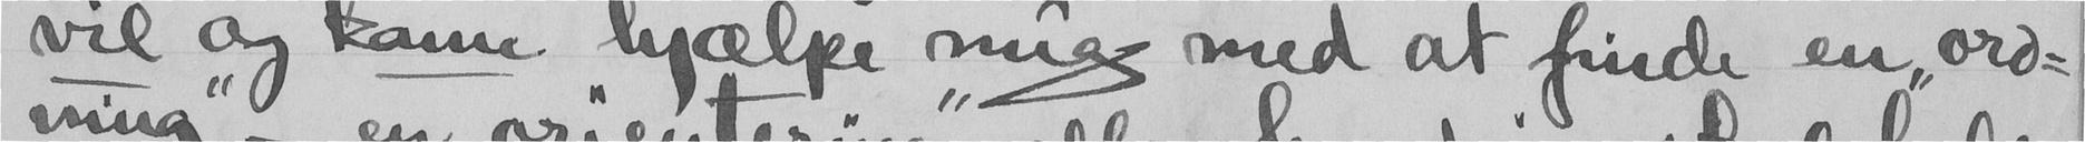vil og kan hjælpe mig med at finde en "ord-
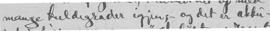mange kuldegrader igjen, - og det er akku-
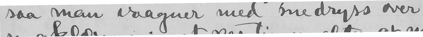saa mam vaagner med snedryss over
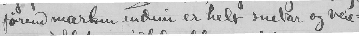førend mærken endnu er helt snebar og veie-
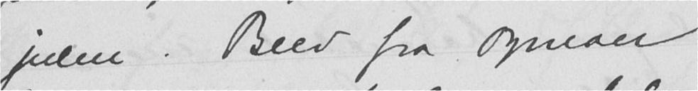julen. Brev fra Ogman
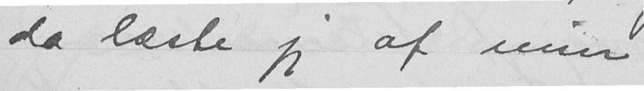da læste jeg af min
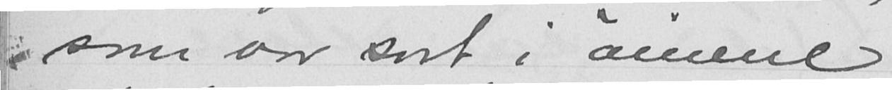som var sort i øinene
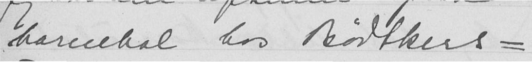barnebal hos Bødtkers-
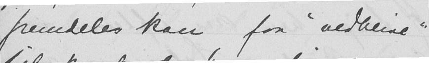fremdeles kan faa "vedblive"
